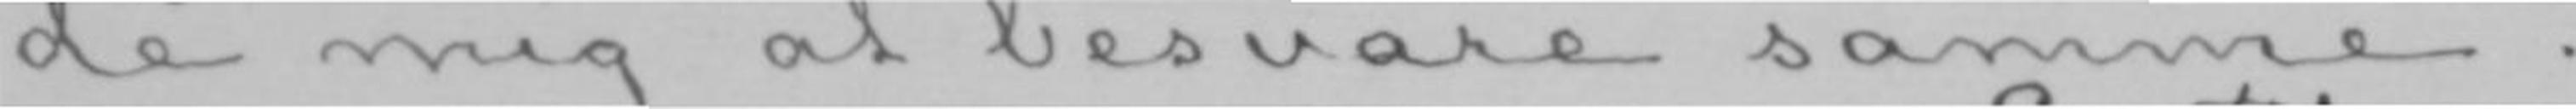de mig at besvare samme.
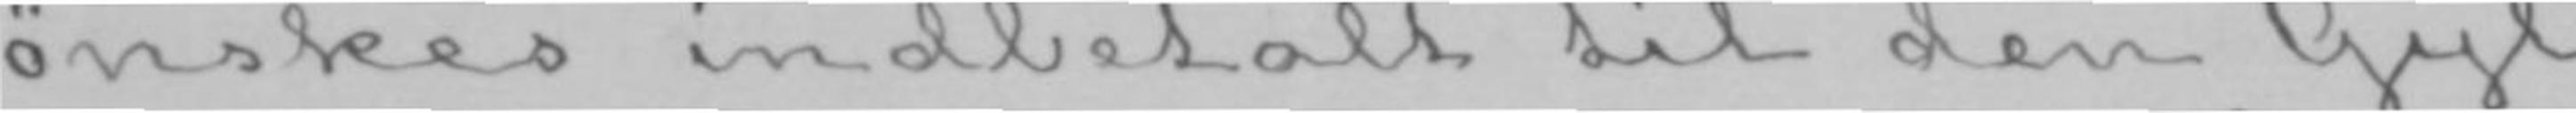ønskes indbetalt til den Gyl-
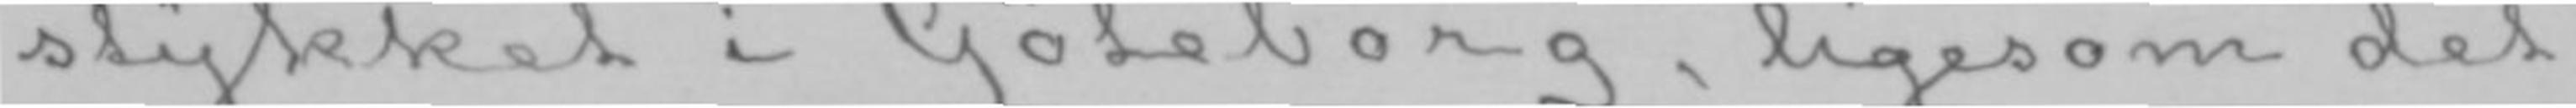stykket i Göteborg, ligesom det
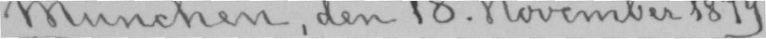München, den 18. November 1879.
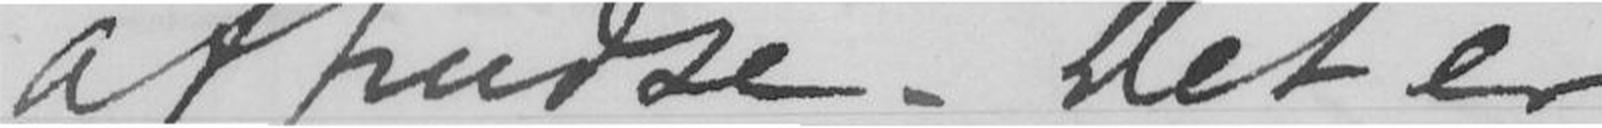afpudse. Det er
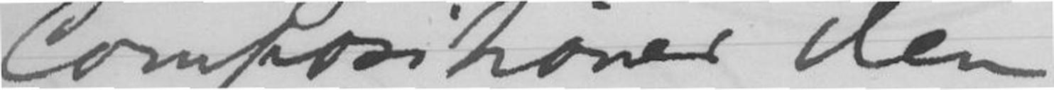Compositioner den
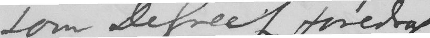som DeGreef foredrag
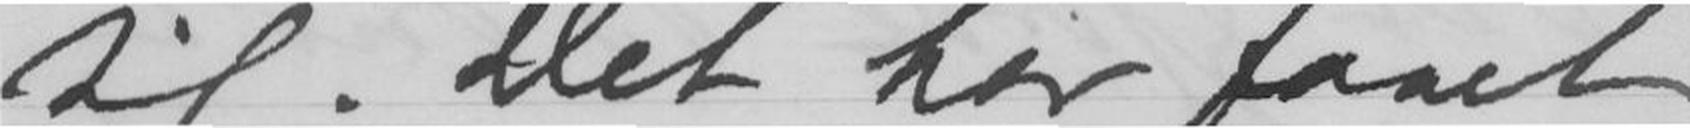sig. Det har faaet
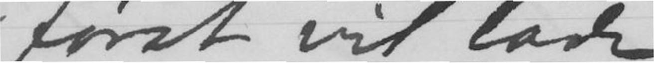jeg først vil lade
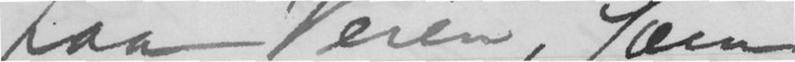paa Veien, som
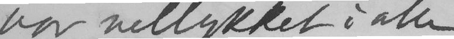var vellykket i alle
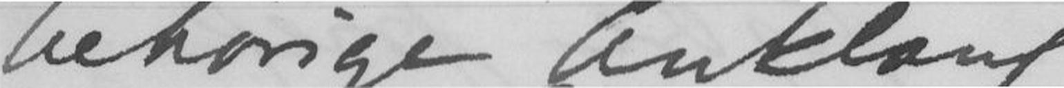behørige Anklang
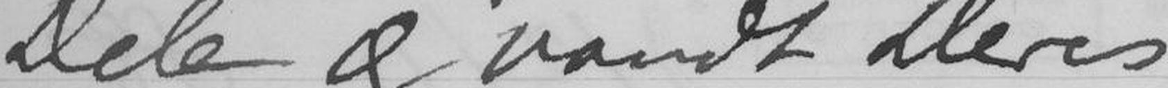Dele og vandt Deres
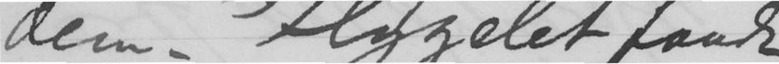Dem. Flygelet fandt
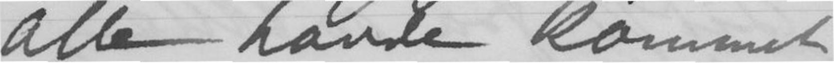alle havde kommet
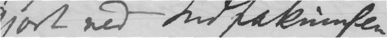gjort ved Indpakningen
